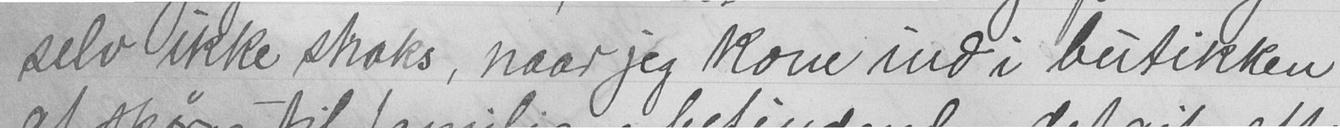selv ikke straks, naar jeg kom ind i butikken
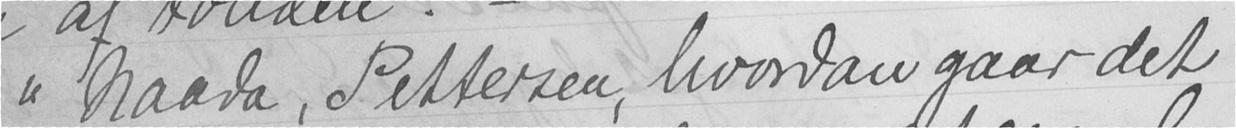"Naada, Pettersen, hvordan gaar det
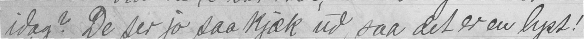idag? De ser jo saa kjæk ud, saa det er en lyst!
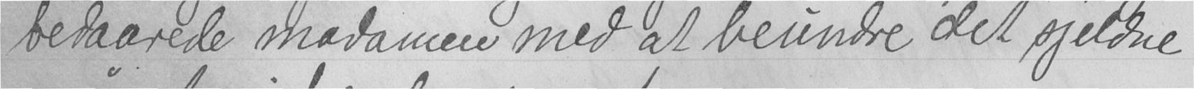bedaarede madamen med at beundre det sjældne
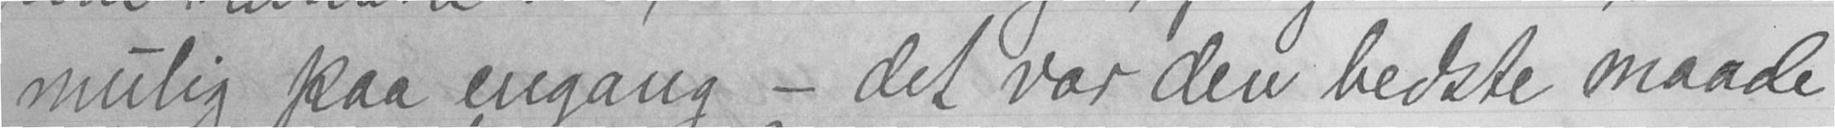mulig paa engang - det var den bedste maade
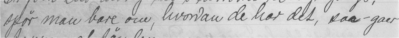spør man bare om, hvordan de har det, saa - gaar
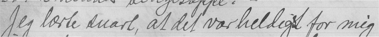Jeg lærte snart, at det var heldigst for mig
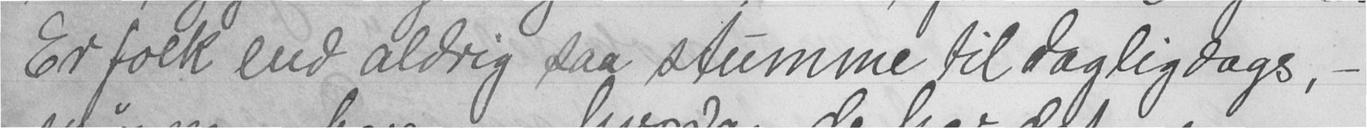Er folk end aldrig saa stumme til dagligdags, -
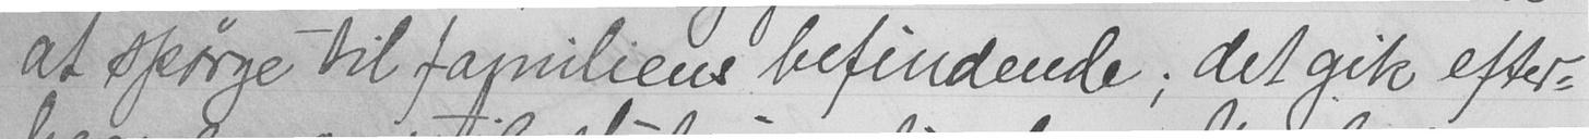at spørge til familiens befindende; det gik efter-
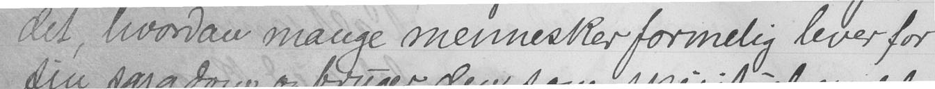det, hvordan mange mennesker formelig lever for
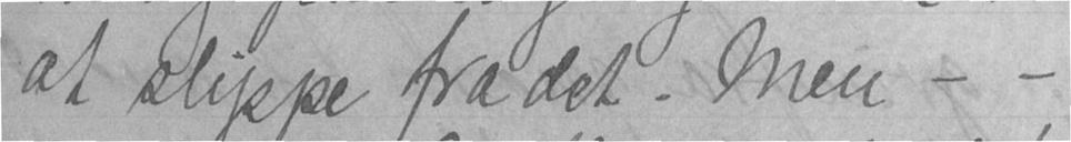at slippe fra det. Men - -
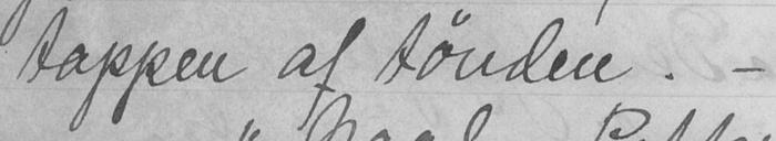tappen af tønden. -
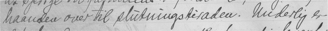haanden over til slutningstiraden. Underlig er
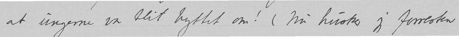at ungerne var blit byttet om! (Nu husker jeg forresten
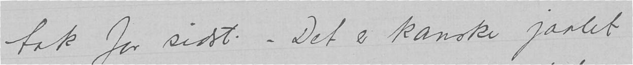tak for sidst. – Det er kanske jaalet
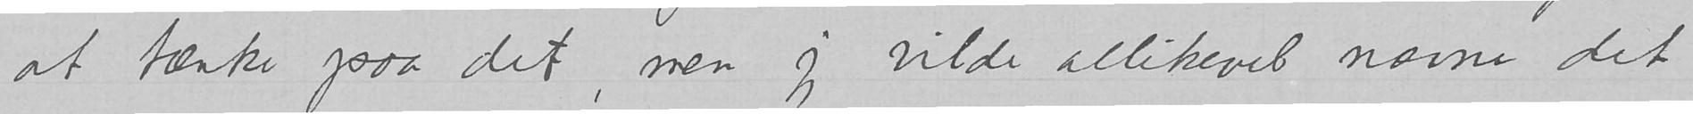at tænke paa det, men jeg vilde allikevel nævne det
